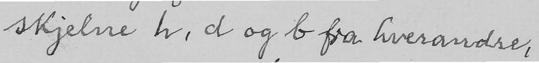skjelne h, d og b fra hverandre,
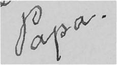Papa.
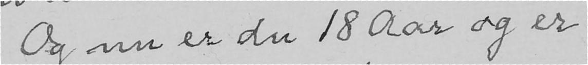Og nu er du 18 Aar og er
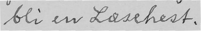bli en Læsehest.
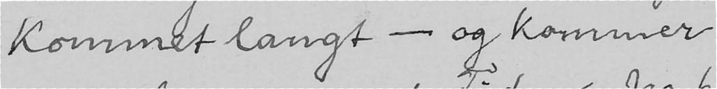kommet langt - og kommer
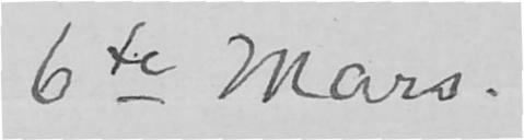6te Mars.
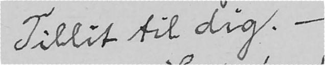Tillit til dig. -
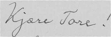Kjære Tore!
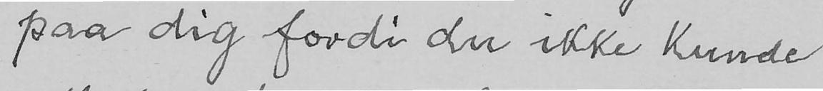paa dig fordi du ikke kunde
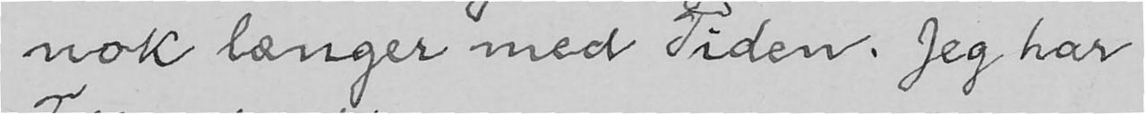nok længer med Tiden. Jeg har
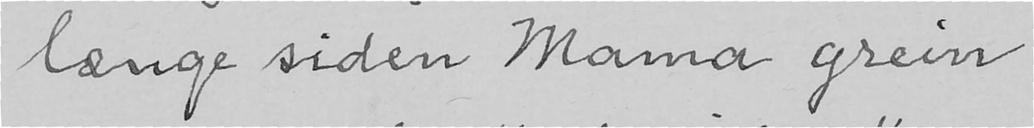længe siden Mama grein
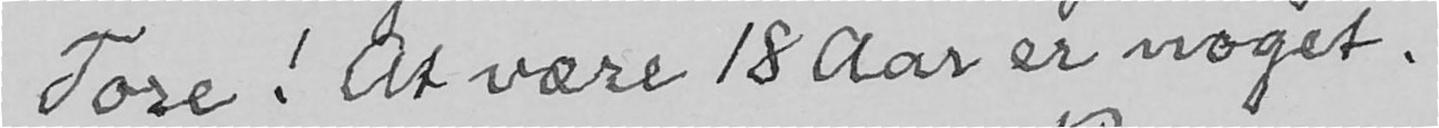Tore! At være 18 Aar er noget.
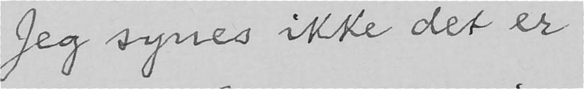Jeg synes ikke det er
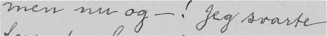men nu og - ! Jeg svarte
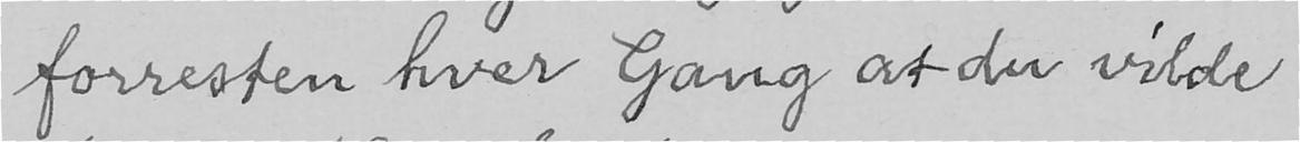forresten hver Gang at du vilde
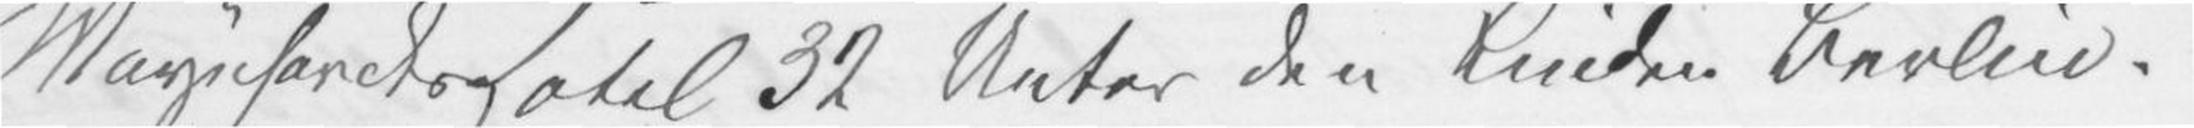Maynhardts Hotel 32 «Unter den Linden» Berlin.
